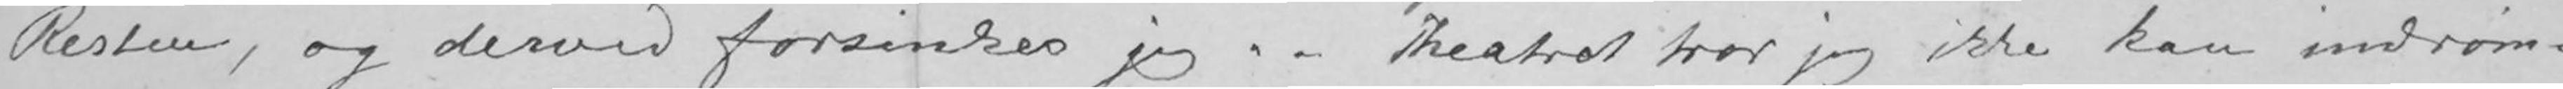Resten, og derved forsinkes jeg.- Theatret tror jeg ikke kan indrøm-
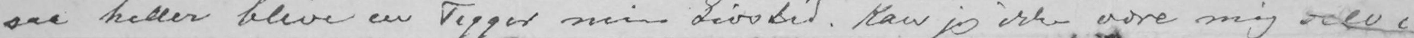saa heller blive en Tigger min Livstid. Kan jeg ikke være mig selv i
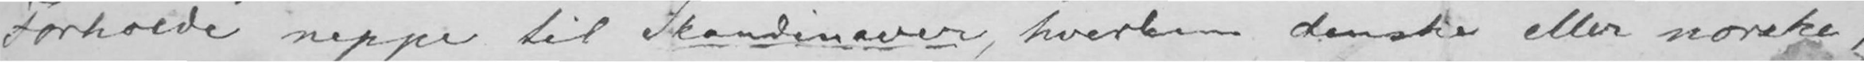Forholde neppe til Skandinaver, hverken danske eller norske;
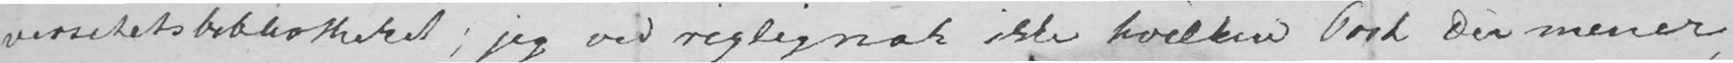versitetsbibliotheket; jeg ved rigtignok ikke hvilken Post Du mener,
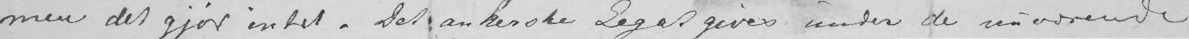men det gjør intet. Det ankerske Legat gives under de nuværende
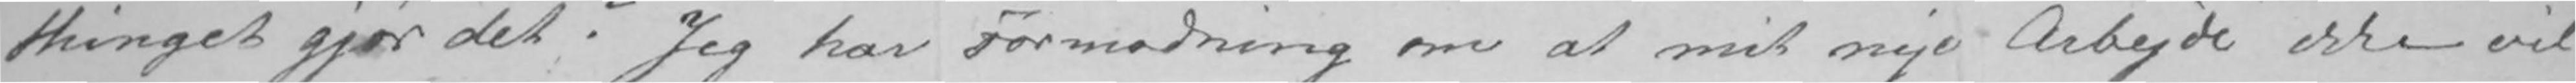thinget gjør det? Jeg har Formodning om at mit nye Arbejde ikke vil
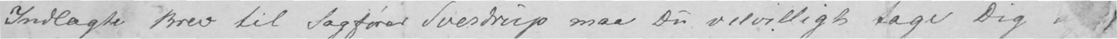Indlagte Brev til Sagfører Sverdrup maa Du velvilligt tage Dig
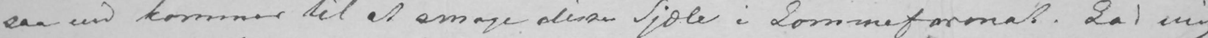saa end kommer til at smage disse Sjæle i Lommeformat. Lad mig
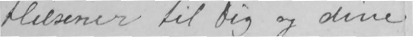Hilsener til Dig og dine.
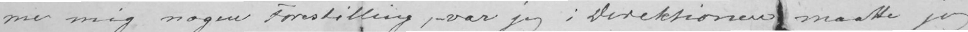me mig nogen Forestilling,- var jeg i Direktionen maatte jeg
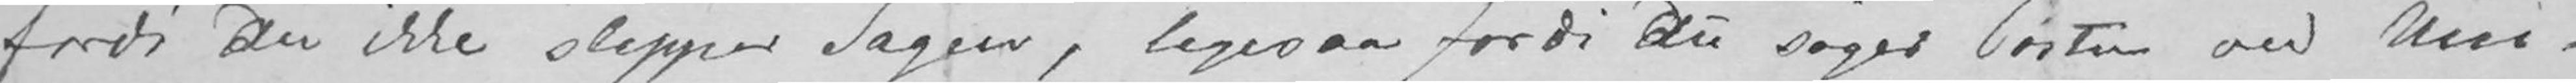fordi Dudu ikke slipper Sagen, ligesaa fordi Dudu søger Posten ved Uni-
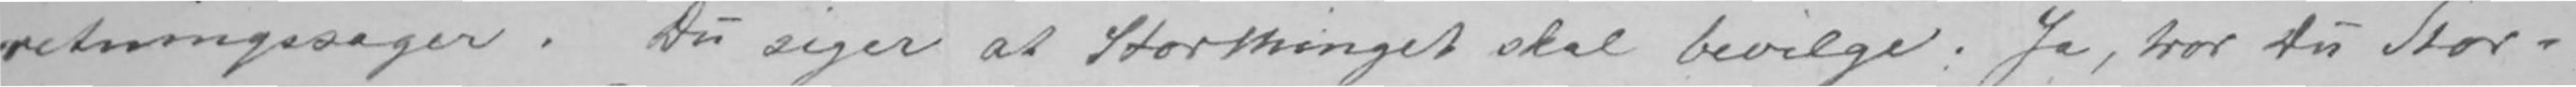retningssager. Du siger at Storthinget skal bevilge. Ja, tror Du Stor-
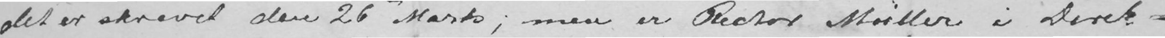det er skrevet den 26de Marts; men er Rector Müller i Direk-
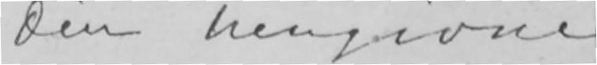Din hengivne
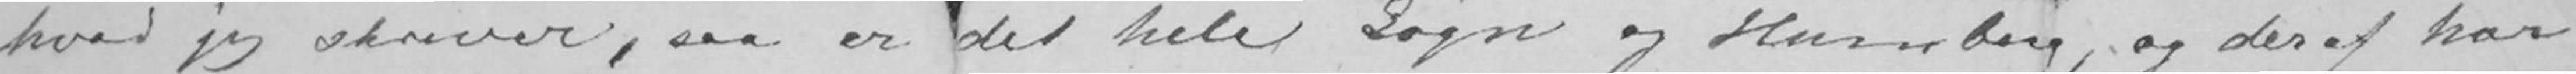hvad jeg skriver, saa er det hele Løgn og Humbug, og deraf har
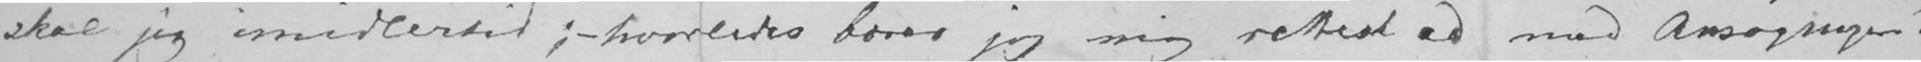skal jeg imidlertid;- hvorledes bærer jeg mig rettest ad med Ansøgningen?
